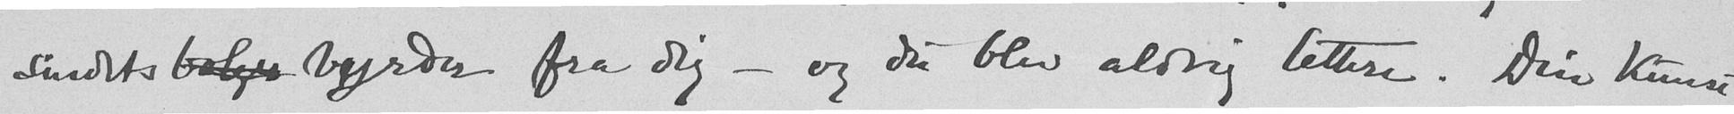sindets balgs byrder fra dig - og du blev aldrig lettere. Din kunst
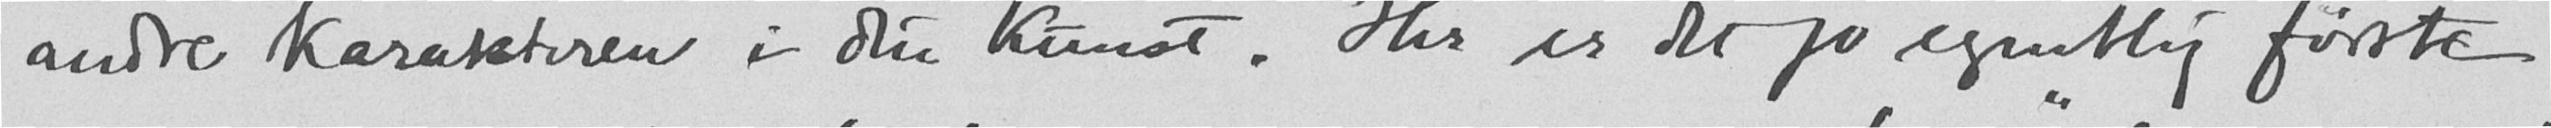andre karakteren i din kunst. Her er det jo egentlig første
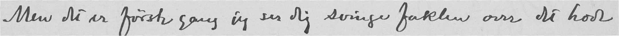Men det er første gang jeg ser dig svinge faklen over det hode
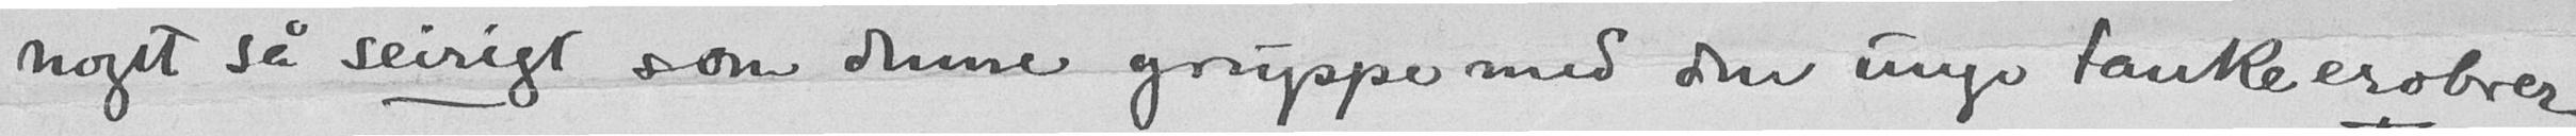noget så seirigt som denne gruppe med den unge tanke erobrer
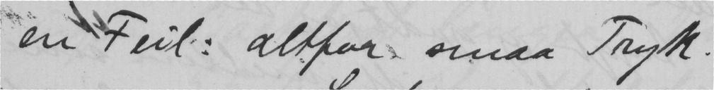en Feil: altfor smaa Tryk.
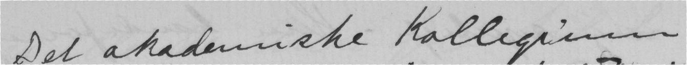Det akademiske Kollegium
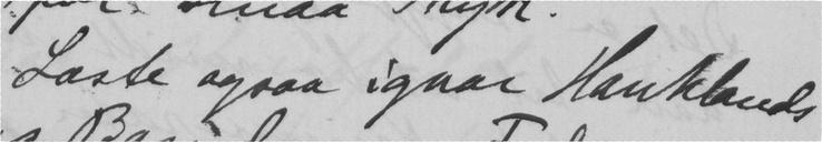Læste ogsaa igaar Hauklands
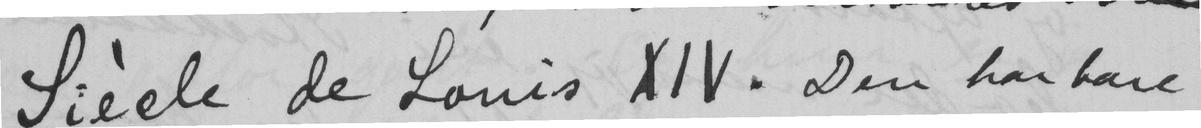Siecle de Louis XIV. Den har bare
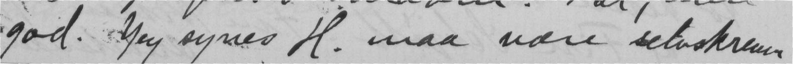god. Jeg synes H. maa være selvskreven
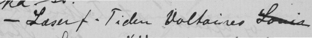- Læser f. Tiden Voltaires
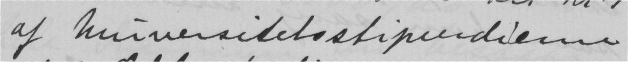af universitetsstipendierne
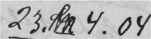23. 4.04
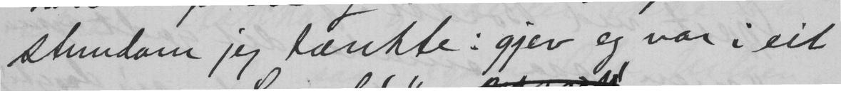stundom jeg tænkte: gjev eg var i eit
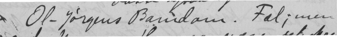Ol- Jørgens Barndom. Fæl; men
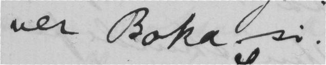ver Boka si.
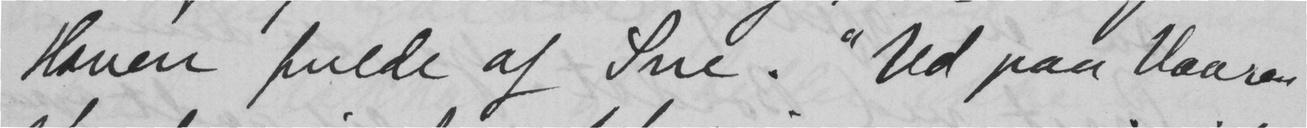Haven fulde af Sne. "Ud paa Vaaren
